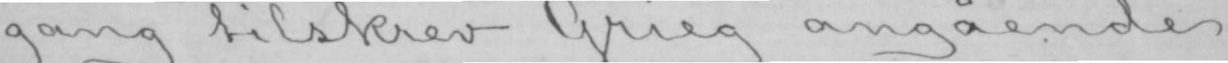gang tilskrev Grieg angående
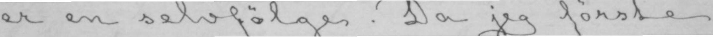er en selvfølge. Da jeg første
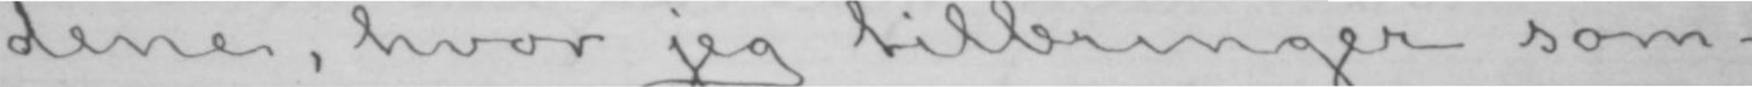dene, hvor jeg tilbringer som-
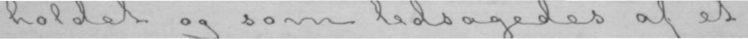holdet og som ledsagedes af et
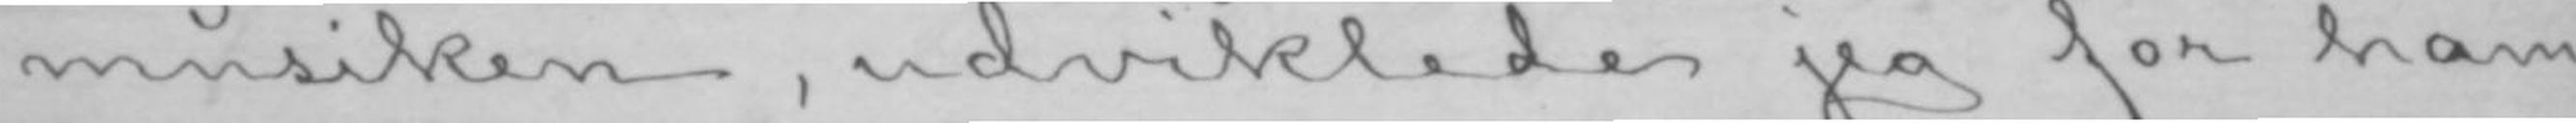musiken, udviklede jeg for ham
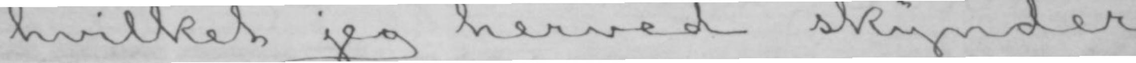hvilket jeg herved skynder
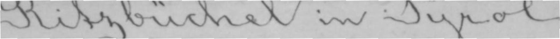Kitzbüchel in Tyrol,
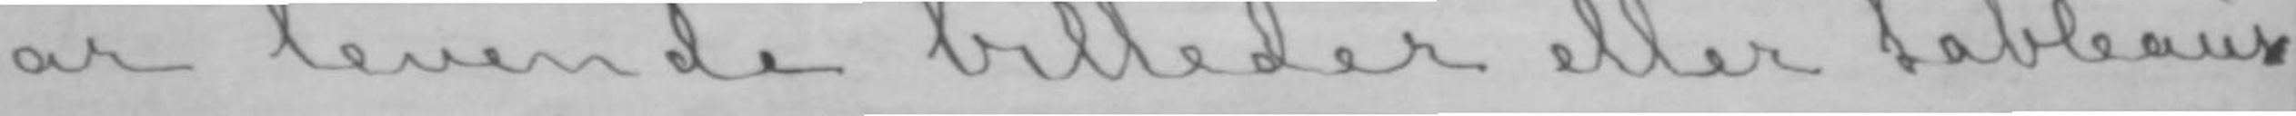par levende billeder eller tableaux
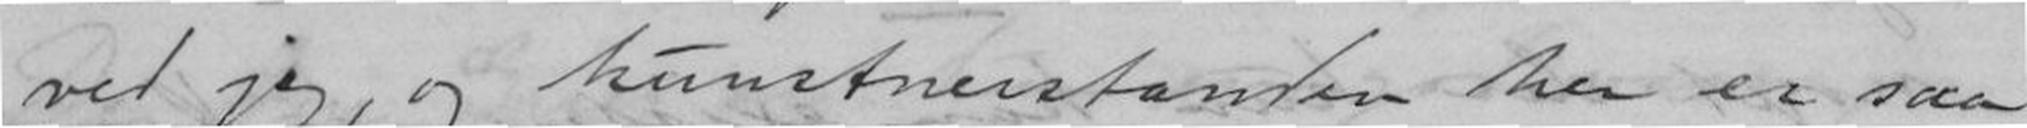ved jeg, og kunstnerstanden her er saa
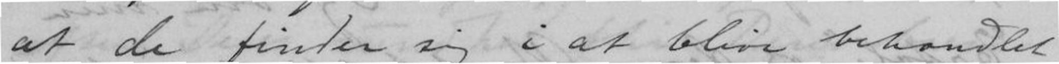at de finder sig at blive behandlet
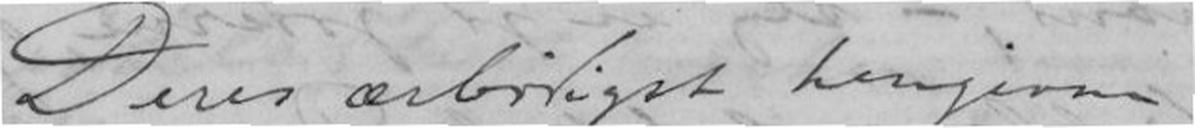Deres ærbødigst hengivne
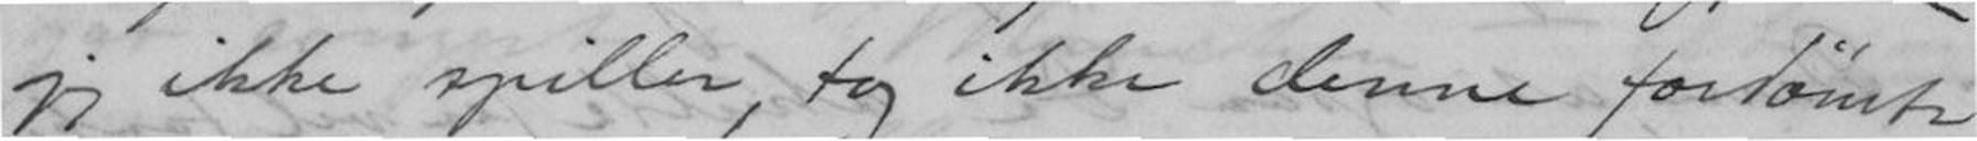jeg ikke spiller, tag ikke denne fordømte
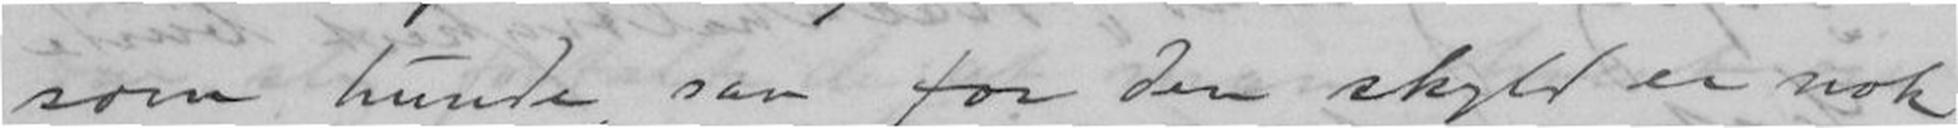som hunde, saa for den skyld er nok
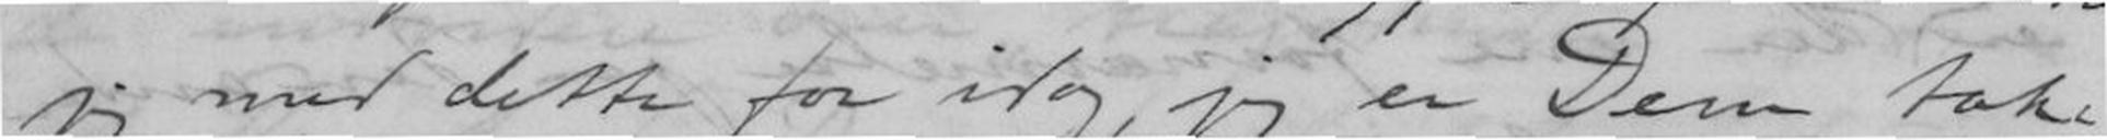jeg med dette for idag, jeg er Dem tak-
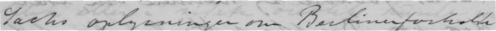Sachs oplysninger om "Berlinerforholde"
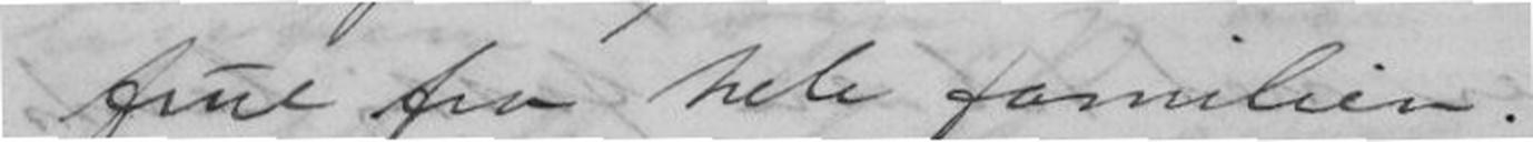frue fra hele familien.
